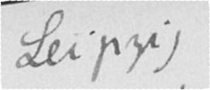Leipzig
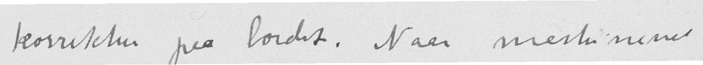korrektur paa bordet. Naar maskinene
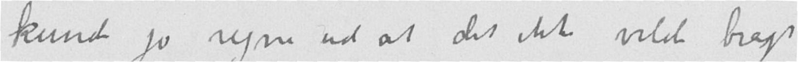kunde jo regne ud at det ikke vilde bragt
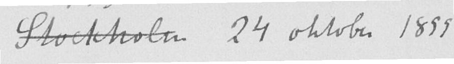Stockholm 24 oktober 1899
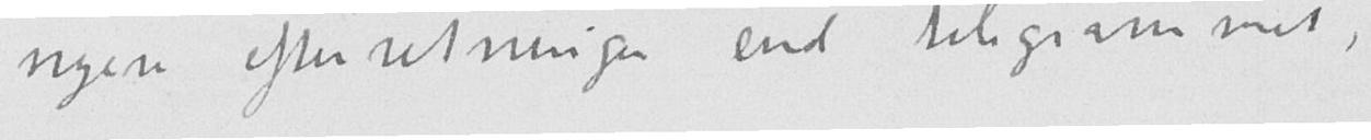nogen efterretninger end telegrammet,
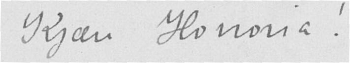Kjære Honoria!
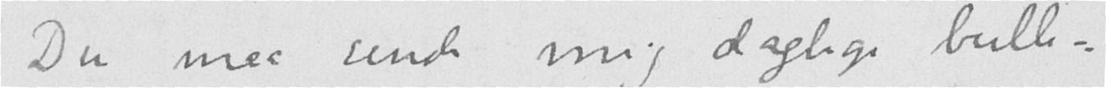Du maa unde mig daglige bulle-
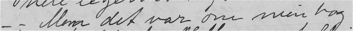-- Men det var om min bog.
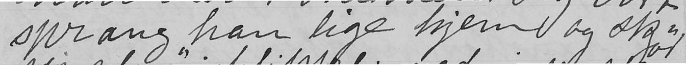sprang han lige hjem og skjød
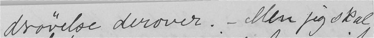drøvelse derover. - Men jeg skal
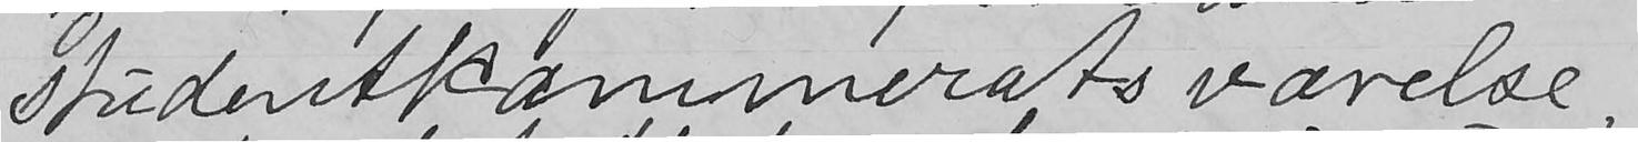studentkammerats værelse,
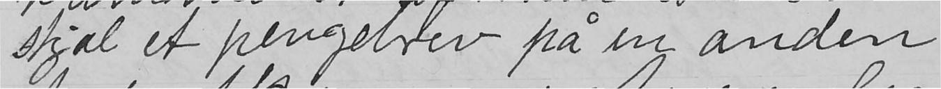stjal et pengebrev på en anden
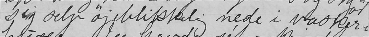sig selv øjeblikkelig nede i vasker-
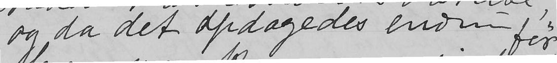og da det opdagedes endnu før
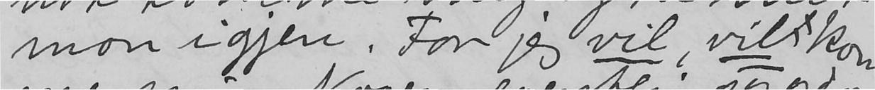mon igjen. For jeg vil, vil kom
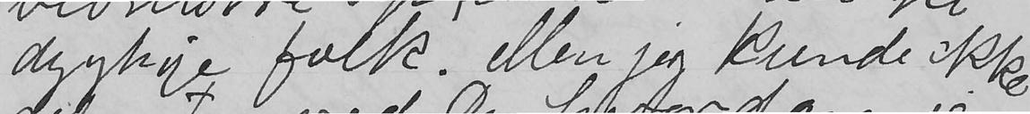dygtige folk. Men jeg kunde ikke
